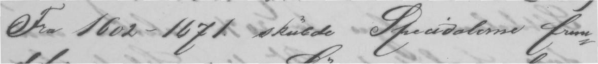Fra 1602-1671 skulde Specidalerne frem-
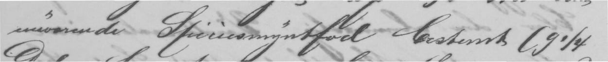enuværende Speciesmyntfod bestemt (9 ¼
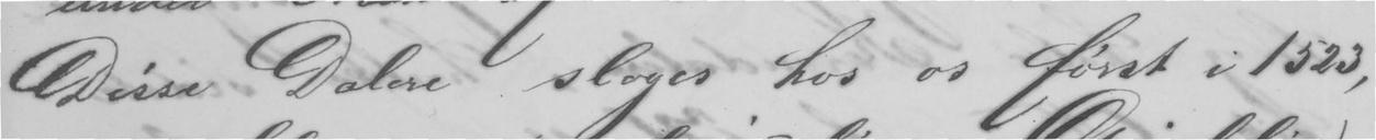Disse Dalere sloges hos os først i 1523,
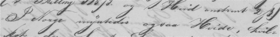I Norge myntedes ogsaa Hvide, hvil-
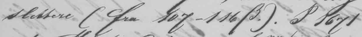slettere (fra 107-116 ẞ.). I 1671
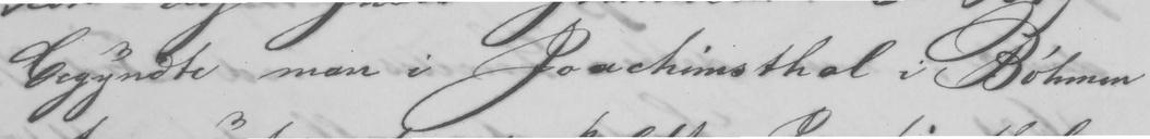begyndte man i Joachimsthal i Bøhmen
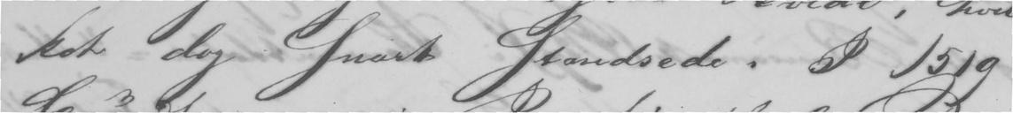ket dog snart standsede. I 1519
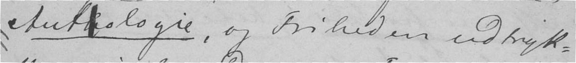Authologie, og Friheden udtryk-
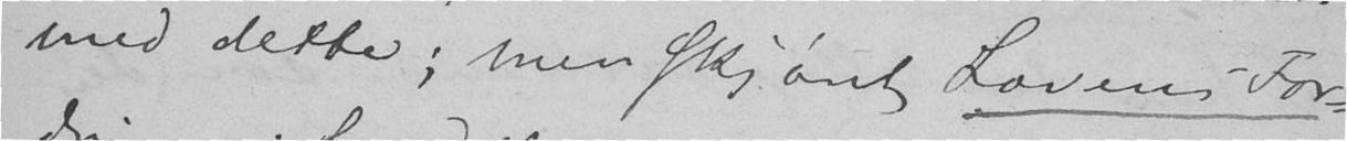med dette; men skjønt Lovens For-
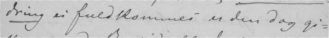dring er fuldkommen er den dog gi-
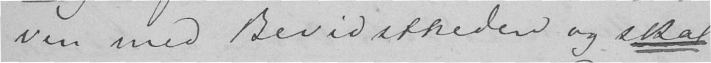ven med Bevidstheden og skal
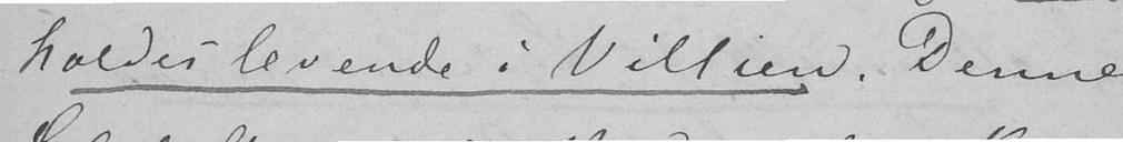holdes levende i Villien. Denne
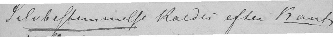Selvbestemmelse kaldes efter Kant
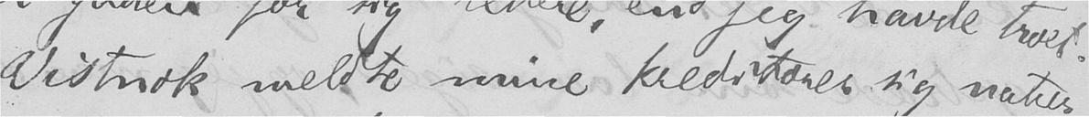Vistnok meldte mine kreditorer sig natur-
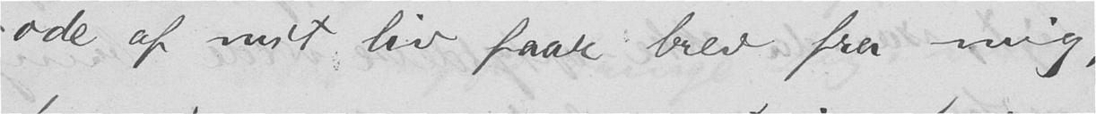ode af mit liv faar brev fra mig,
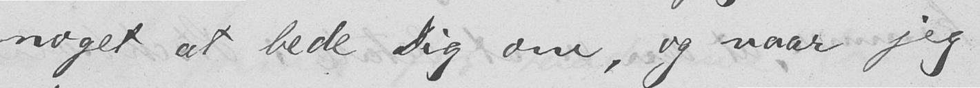noget at bede Dig om, og naar jeg
